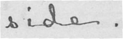side.
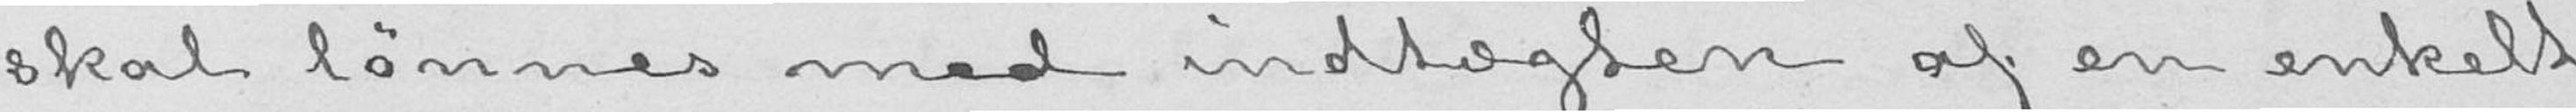skal lønnes med indtægten af en enkelt
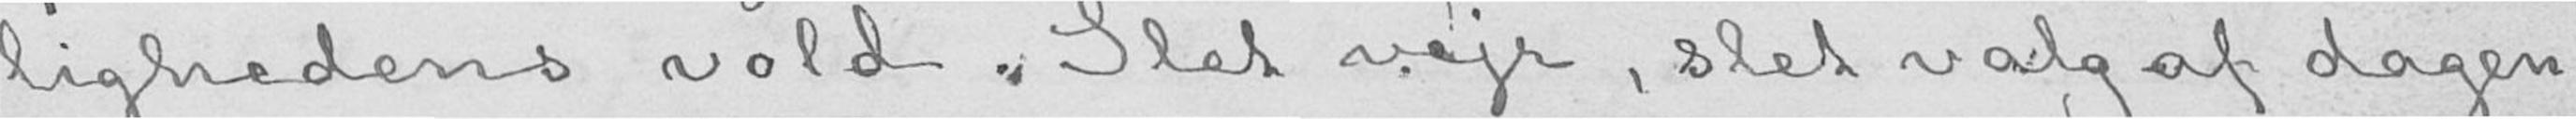lighedens vold. Slet vejr, slet valg af dagen,
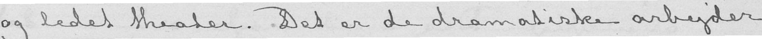og ledet theater. Det er de dramatiske arbejder
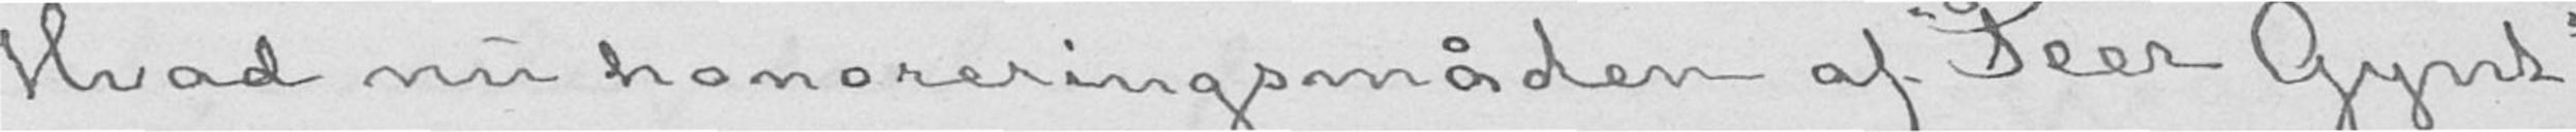Hvad nu honoreringsmåden af «Peer Gynt»
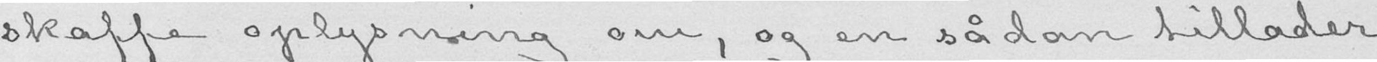skaffe oplysning om, og en sådan tillader
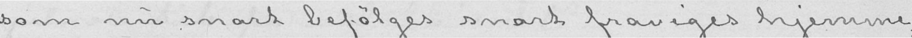som nu snart befølges snart fraviges hjemme,
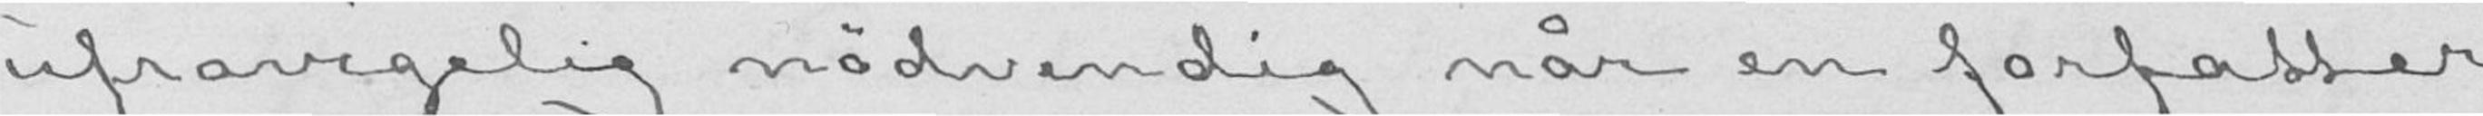ufravigelig nødvendig når en forfatter
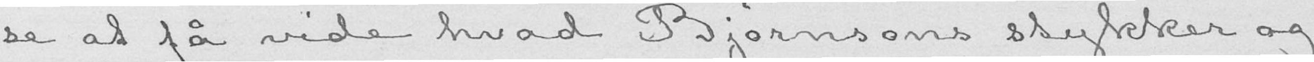se at få vide hvad Bjørnsons stykker og
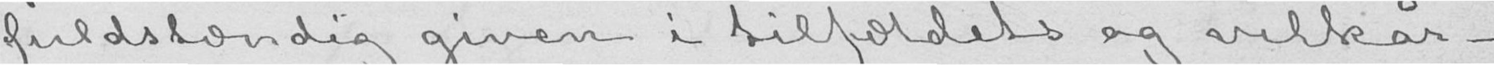fuldstændig given i tilfældets og vilkår-
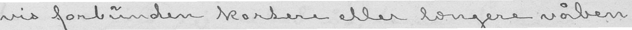vis forbunden kortere eller længere våben-
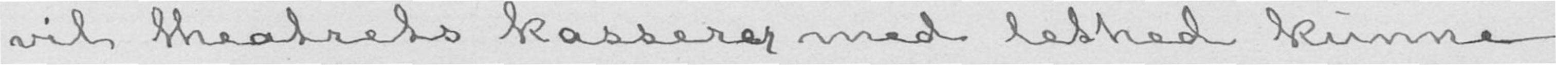vil theatrets kasserer med lethed kunne
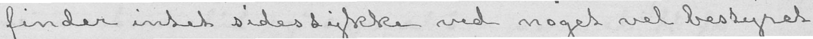finder intet sidestykke ved noget vel bestyret
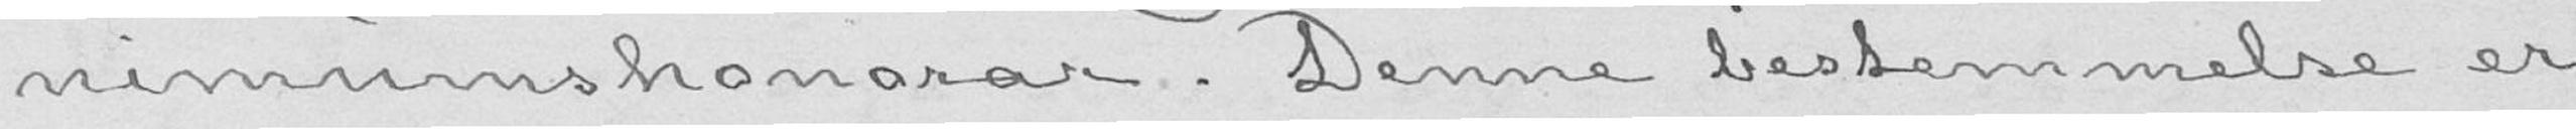nimumshonorar. Denne bestemmelse er
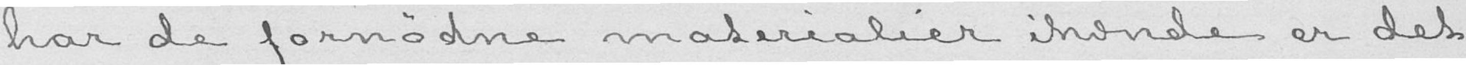har de fornødne materialier ihænde er det
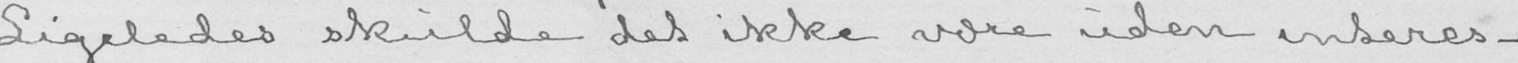Ligeledes skulde det ikke være uden interes-
